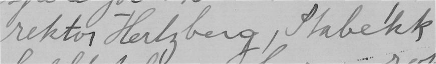rektor Hertzberg, Stabekk
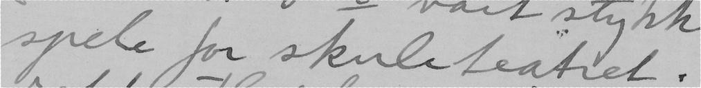spele for skuleteatret,
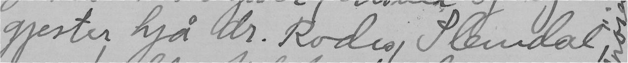gjester hjå dr. Rodes, Slemdal,
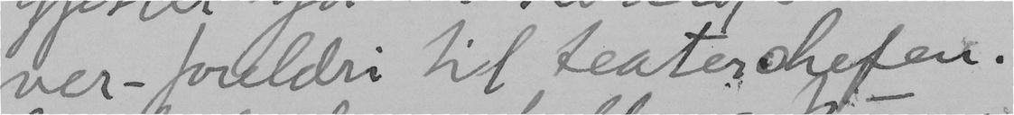ver-foreldri til teaterchefen.
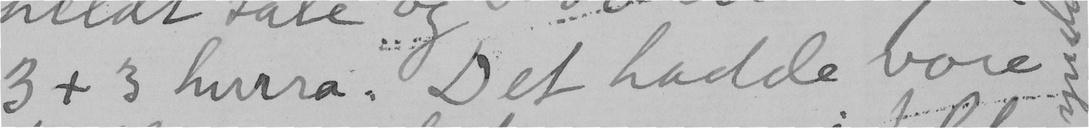3 + 3 hurra. Det hadde vore
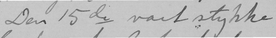Den 15de vart stykke
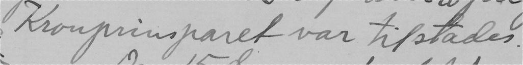Kronprinsparet var tilstades.
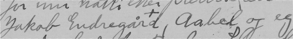Jakob Endregård, Aabel og eg
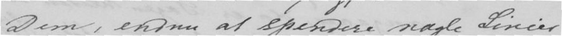Dem, endnu at spandere nogle Linier
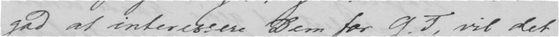god at interessere Dem for G. T, vil det
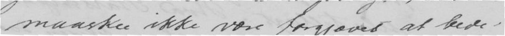maaske ikke være forgjæves at bede
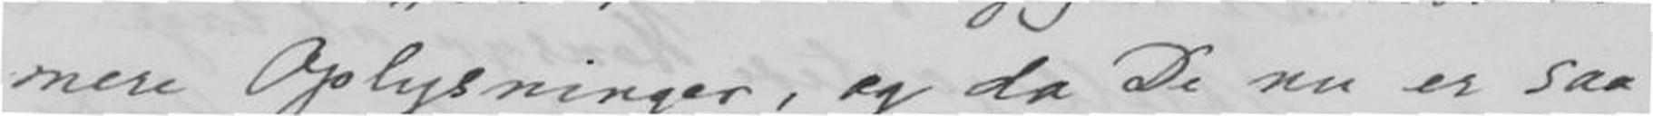mere Oplysninger, og da De nu er saa
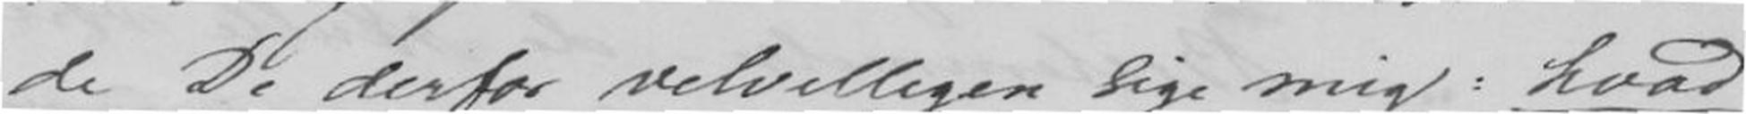de De derfor velvilligen Sige mig: hvad
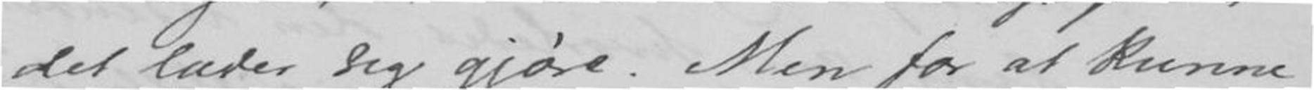det lader sig gjøre. Men for at kunne
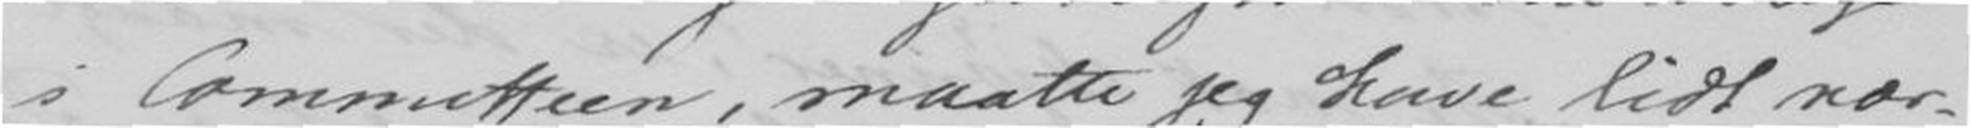i Committeen, maatte jeg have lidt nær-
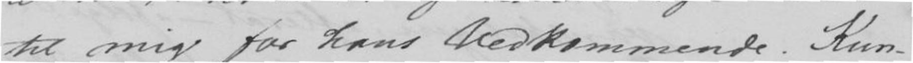til mig for hans Vedkommende. Kun-
